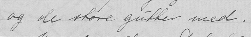og de store gutter med.
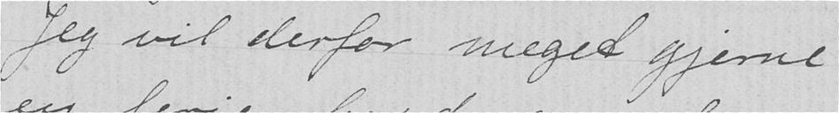Jeg vil derfor meget gjerne
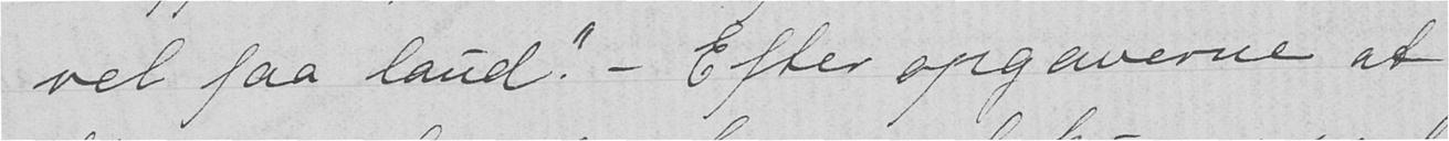vel faa laud." - Efter opgaverne at
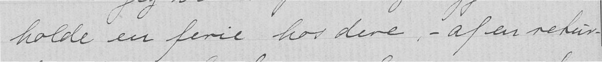holde en ferie hos dere, - af en retur-
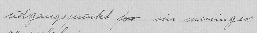udgangspunkt for sin meninger
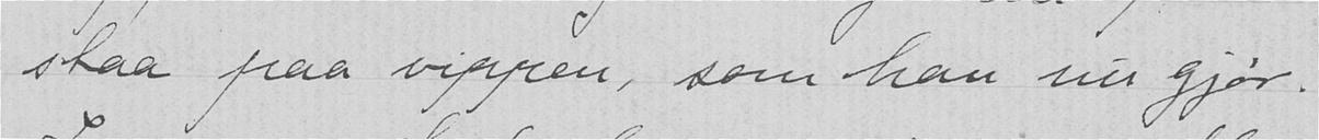staa paa vippen, som han nu gjør.
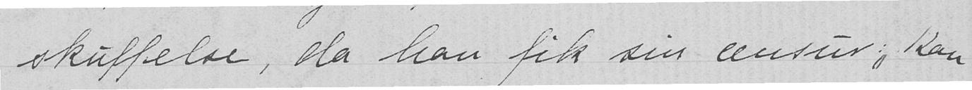skuffelse, da han fik sin censur: "Kan
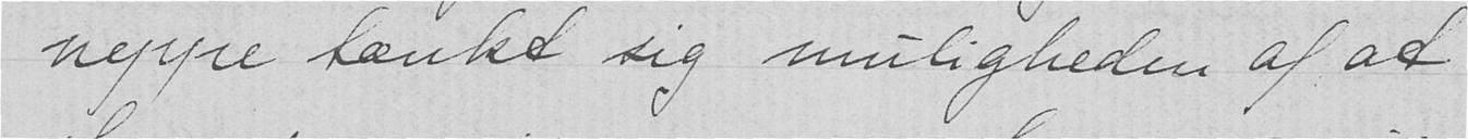neppe tænkt sig muligheden af at
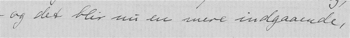- og det blir nu en mere indgaaende,
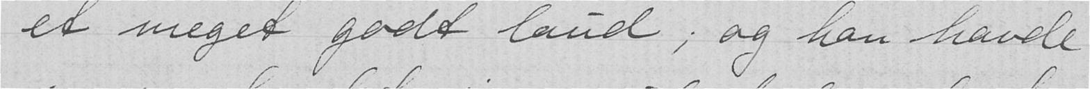et meget godt laud; og han havde
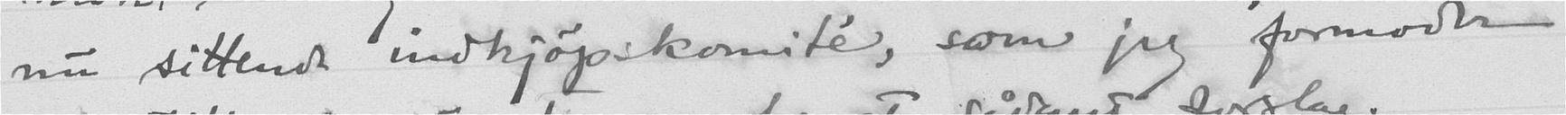nu sittende indkjøpskomité, som jeg formoder
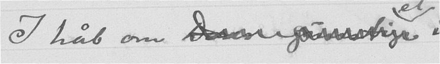I håb om Deres gunstige
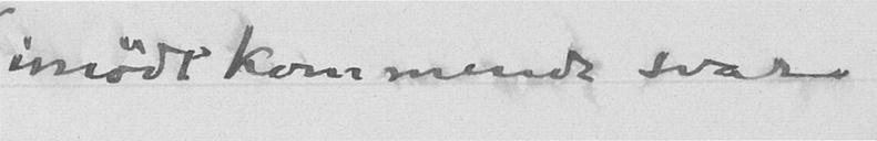imødt kommende svar
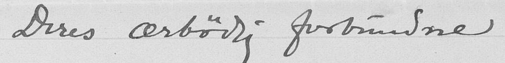Deres ærbødig forbundne
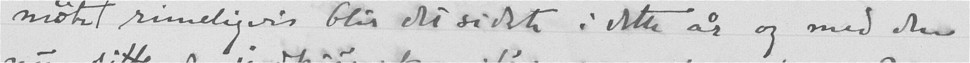møtet rimeligvis blir det sidste i dette år og med den
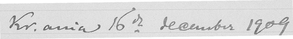Kr.ania 16de december 1909.
